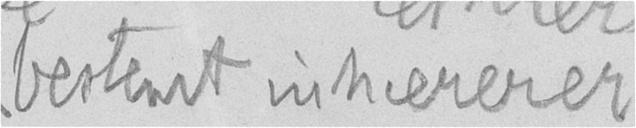bestemt nihiererer
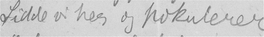Sidde vi her og pokulerer
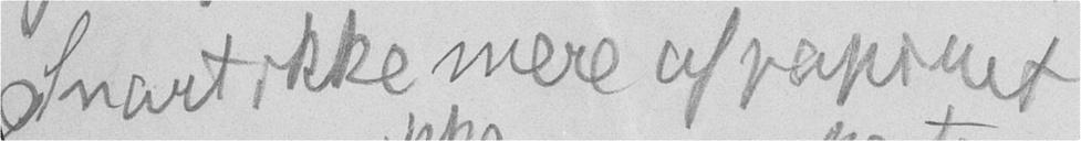Snart ikke mere af papiret
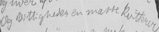og vittigheder en masse kvitterer
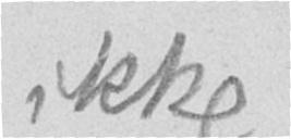ikke
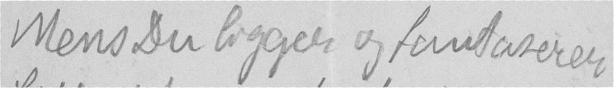Mens Du ligger og fantaserer
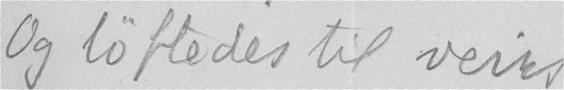Og løftedes til veirs,
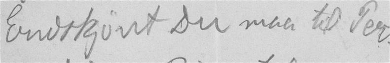Endskjønt Du maa til Pers,
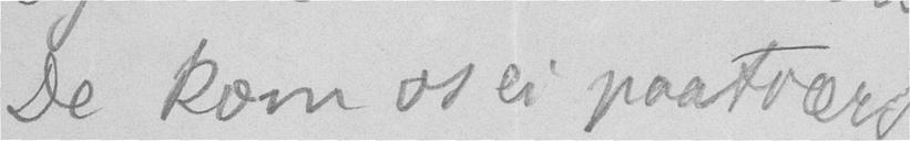De kom os ei paatværs.
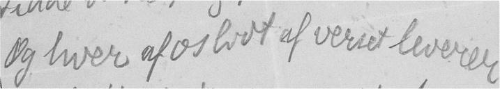Og hver af os lidt af verset leverer,
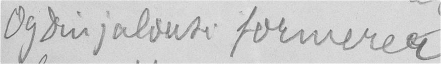Og Din jalusi formerer;
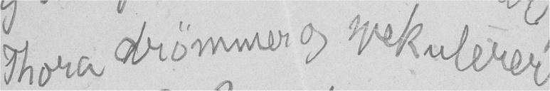Thora drømmer og spekulerer,
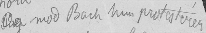Dog mod Bach hun protesterer,
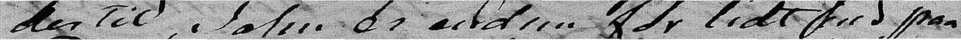der til, John er endnu for lidt ind paa
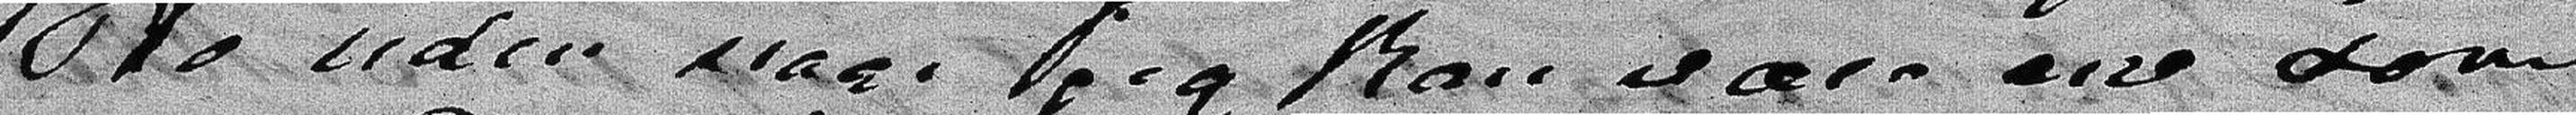Ro uden naar jeg kan være ene som
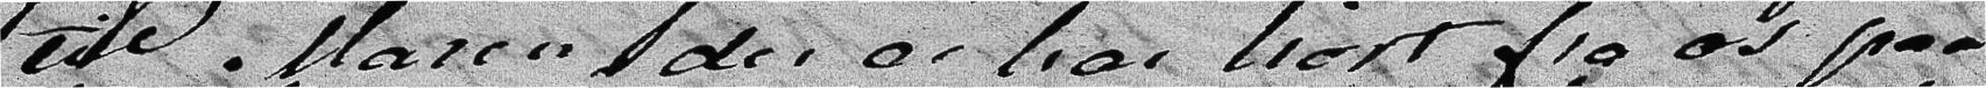til Maren, der ei har hørt fra os paa
